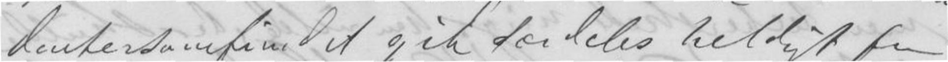dertersamfundet gik særdeles heldigt for
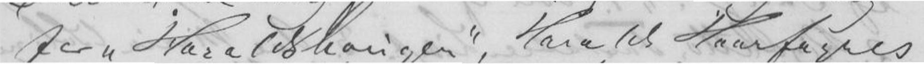for Haraldshougen, Harald Haarfagres
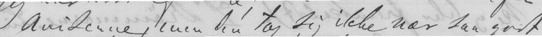Aviserne, men den tog sig ikke nær saa godt
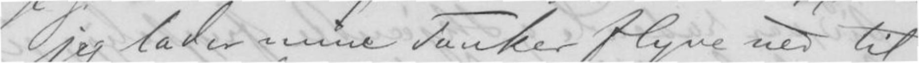jeg lader mine Tanker flyve ned til
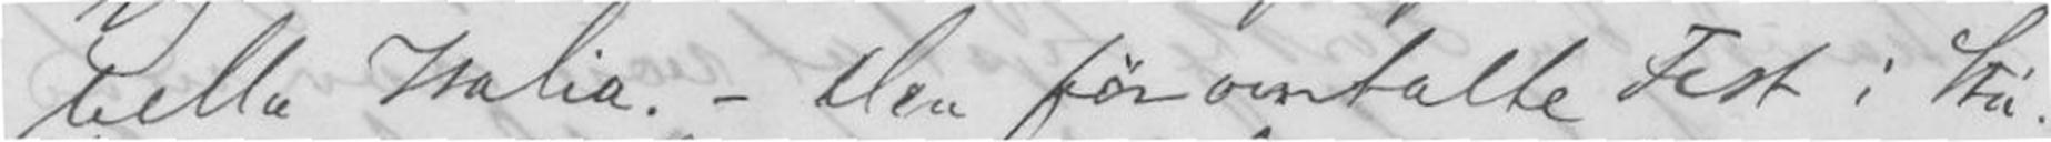bella Italia. - Den føromtalte Fest i Stu-
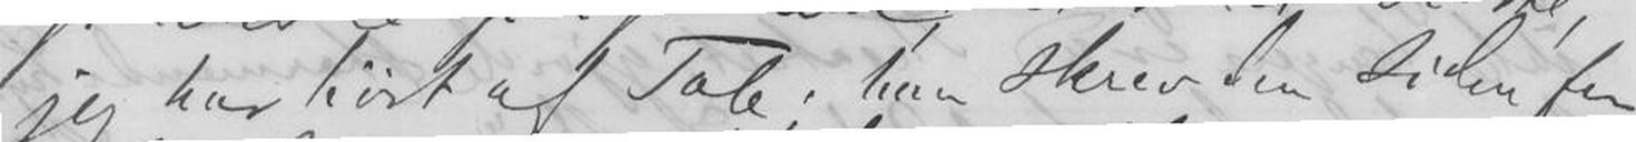jeg har hørt af Tale; han skrev den siden for
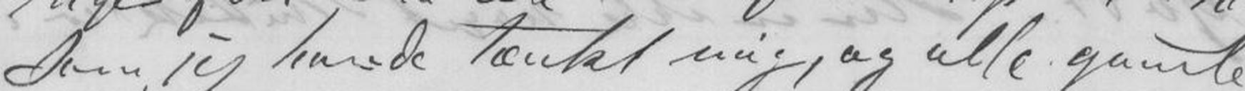som jeg havde tænkt mig, og alle gamle
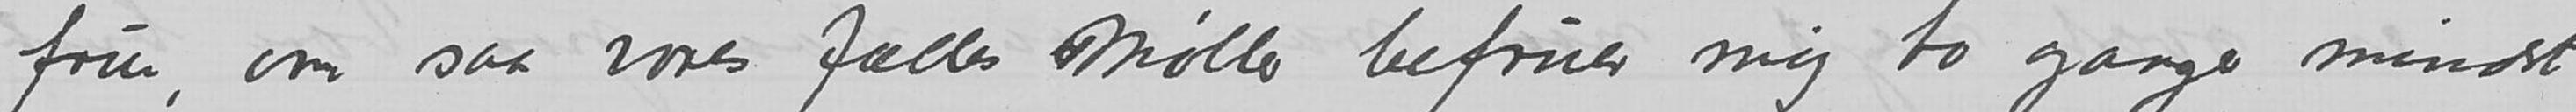frue, om saa vores fælles Møller befruer mig to ganger mindst
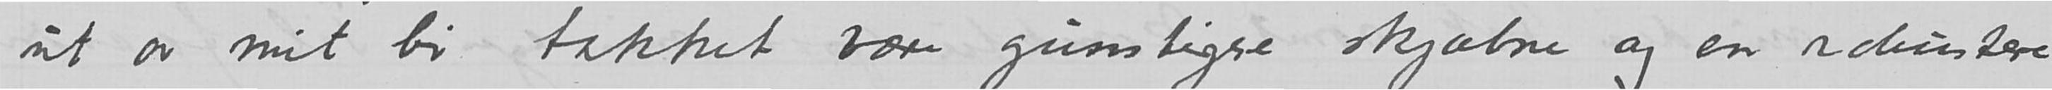ut av mit liv takket være gunstigere skjæbne og en robustere
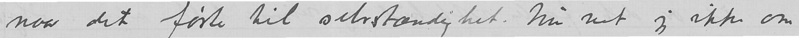naar det førte til selvstændighet. Nu vet jeg ikke om
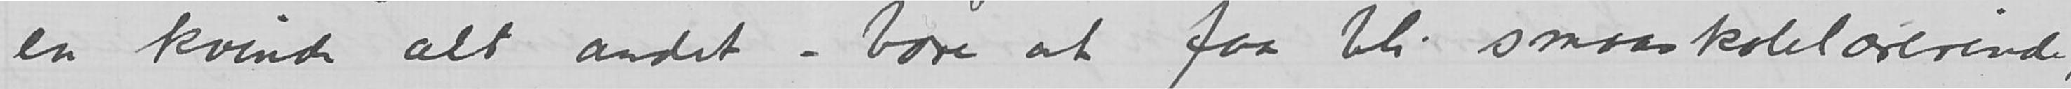en kvinde alt andet - bare at faa bli smaaskolelærerinde,
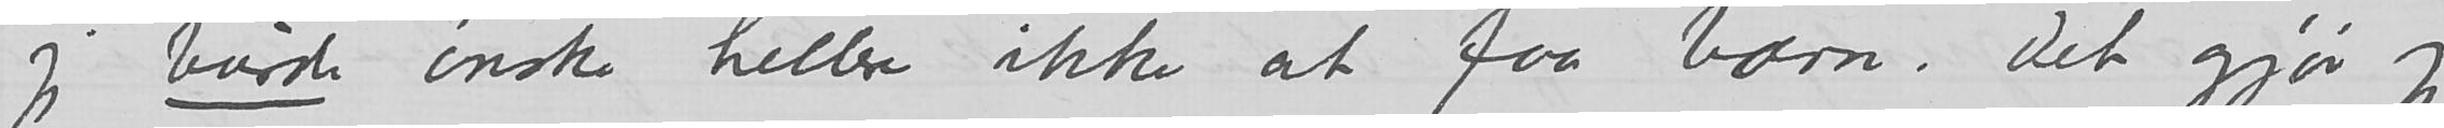jeg burde ønske hellere ikke at faa barn. Det gjør jeg
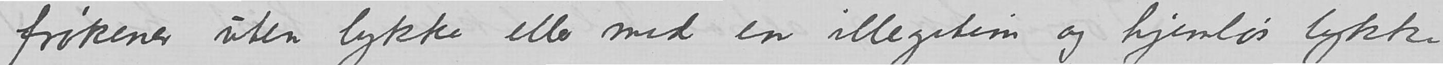frøkener uten lykke eller med en illegitim og hjemløs lykke -
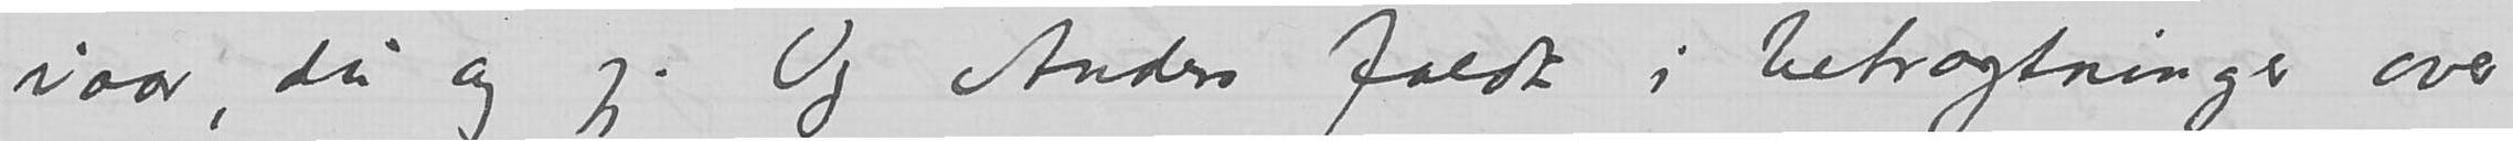iaar, du og jeg. Og Anders faldt i betragtninger over
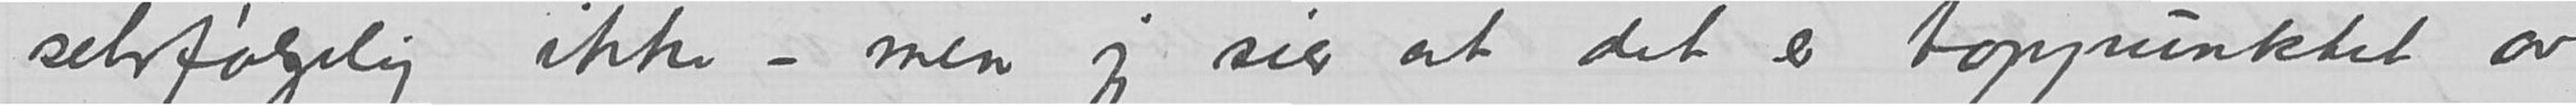selvfølgelig ikke - men jeg sier at det er toppunktet
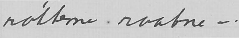røtterne raatne -.
